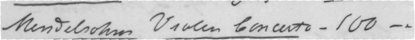Mendelsohn Violin Concert - 100 - " -
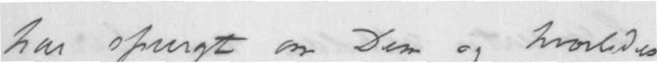har spurgt om Dem og hvorledes
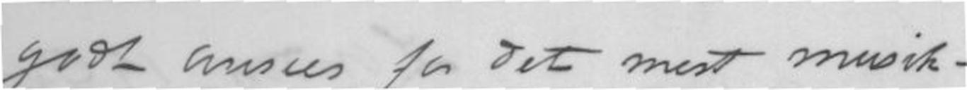godt ansees for det mest musik-
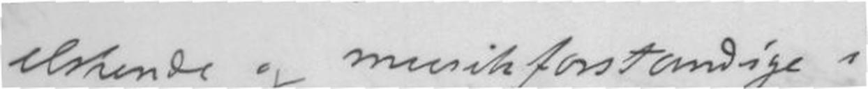elskende og musikforstandige i
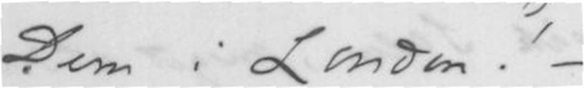Dem i London! -
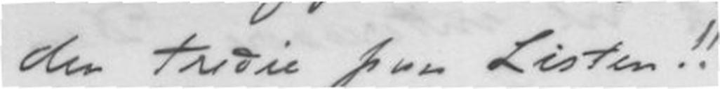den tredie paa Listen!!
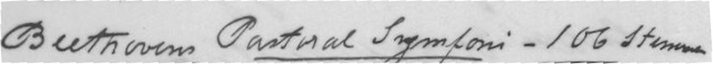Beethovens Pastoral Symfoni - 106 stemmer
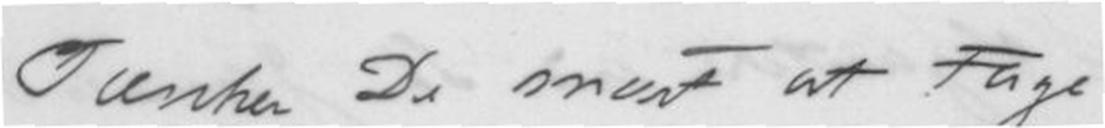Tænker De snart at tage en
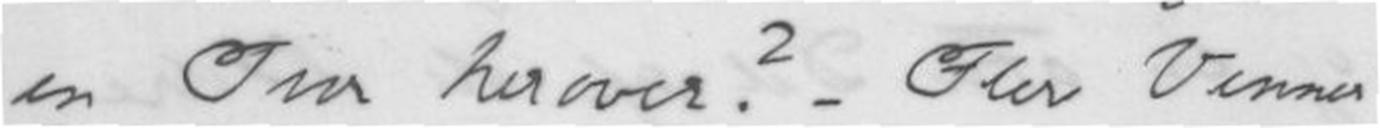Tur herover? - Fler Venner
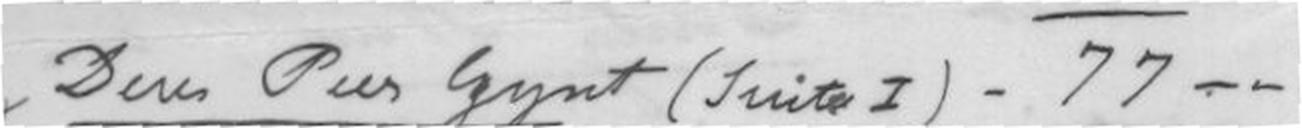og Deres Peer Gynt (Suite I)- 77 - " -
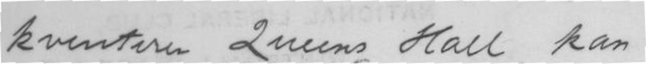kventerer Queens Hall, kan
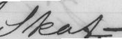Skat. -
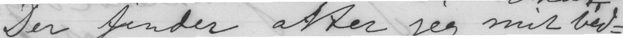Der finder atter jeg mit bed-
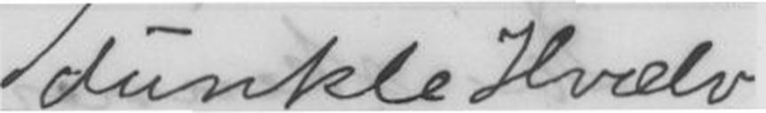dunkle Hvælv,
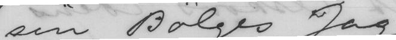sin Bølges Jag.
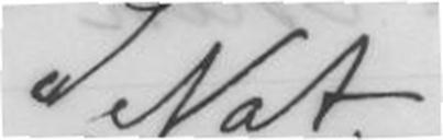Nat
